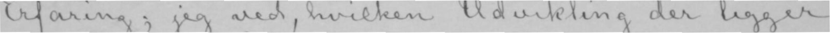Erfaring; jeg ved, hvilken Udvikling der ligger
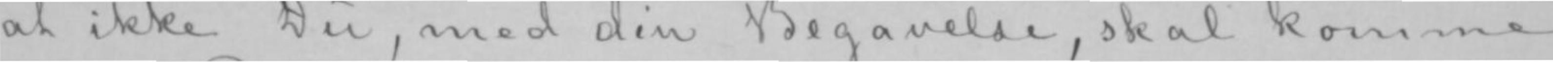at ikke Du, med din Begavelse, skal komme
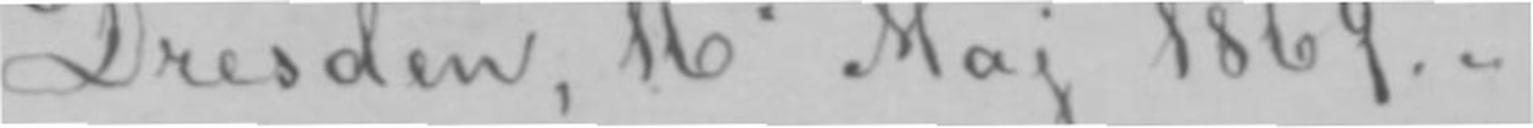Dresden, 16de Maj 1869.-
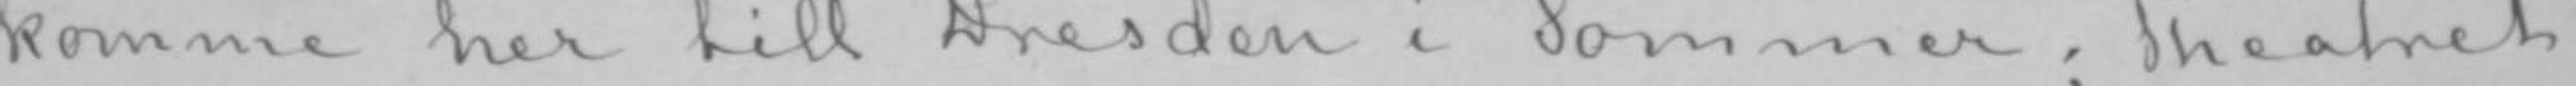komme her till Dresden i Sommer; Theatret
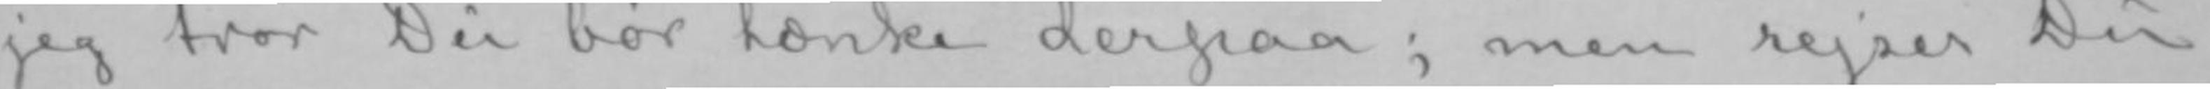jeg tror Du bør tænke derpaa; men rejser Du
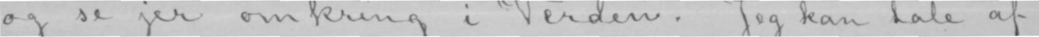og se jer omkring i Verden. Jeg kan tale af
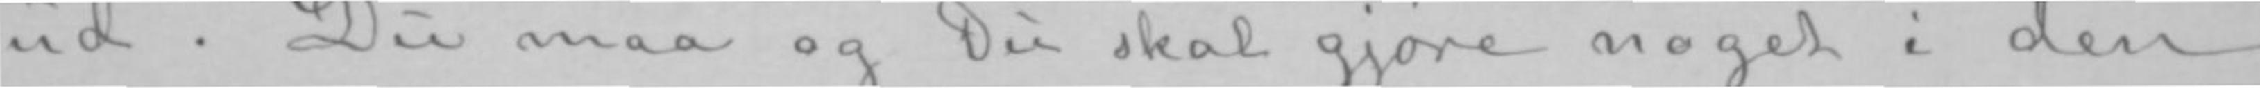ud. Du maa og Du skal gjøre noget i den
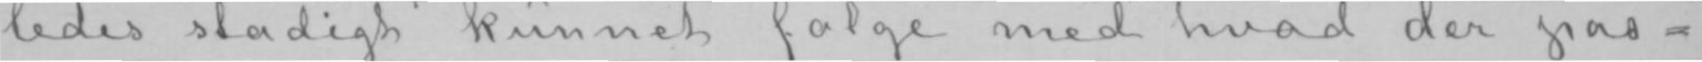ledes stadigt kunnet følge med hvad der pas-
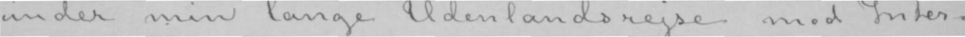under min lange Udenlandsrejse med Inter-
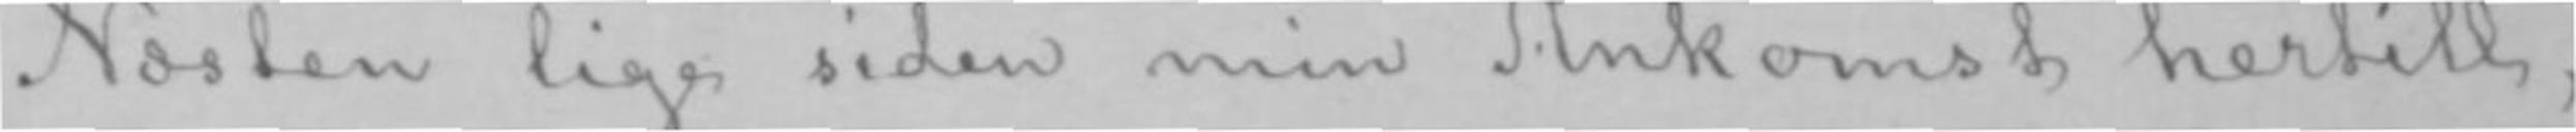Næsten lige siden min Ankomst hertill,
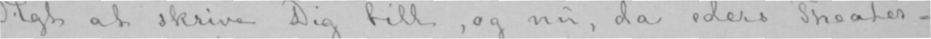Agt at skrive Dig till, og nu, da eders Theater-
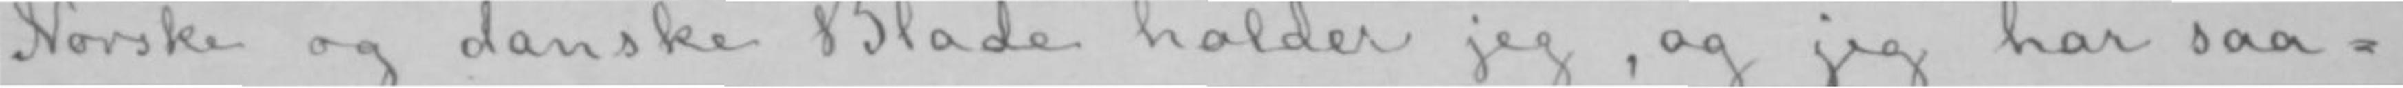Norske og danske Blade holder jeg, og jeg har saa-
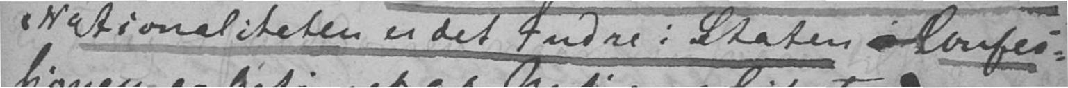Nationaliteten er det Indre i Staten Confes-
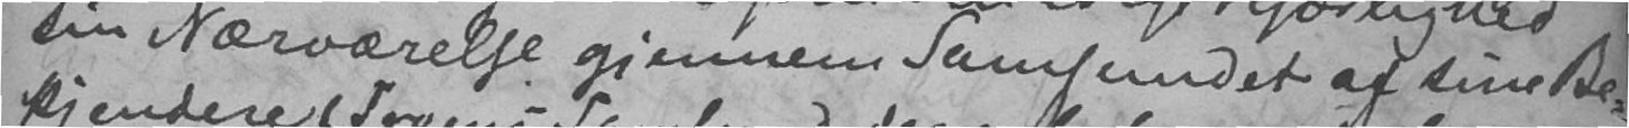sin Nærværelse gjennem Samfundet af sine Be-
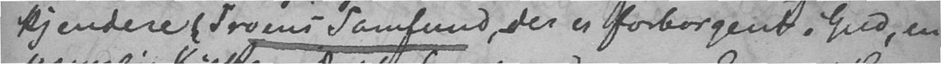kjendere (Troens Samfund, der er forborgent. Gud, en
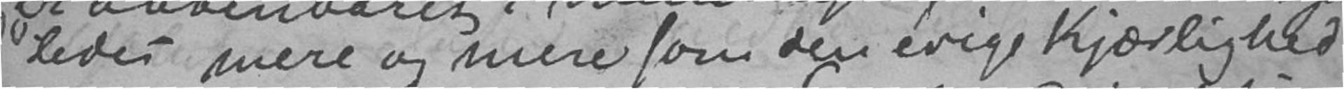ledes mere og mere som den evige Kjærlighed
